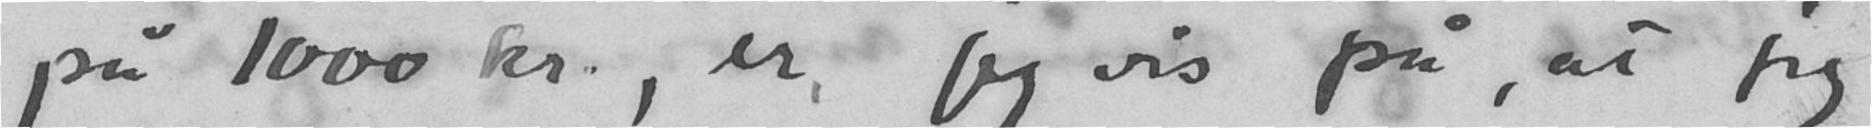på 1000 kr., er jeg vis på, at jeg
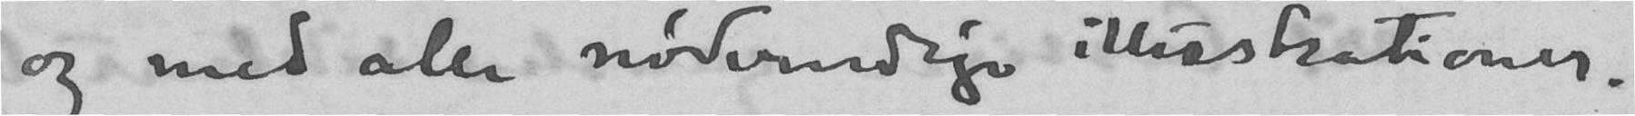og med alle nødvendige illustrationer.
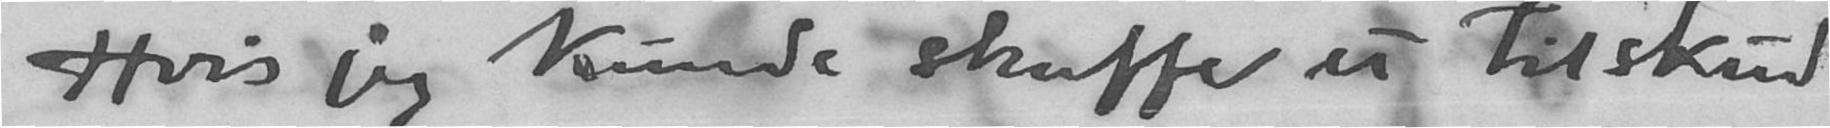Hvis jeg kunde skaffe et tilskud
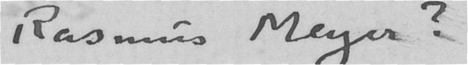Rasmus Meyer?
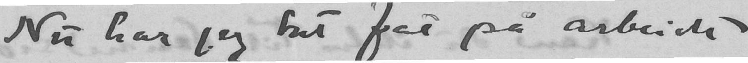Nu har jeg tat fat på arbeidet
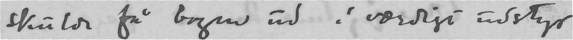skulde få bogen ud i værdigt udstyr
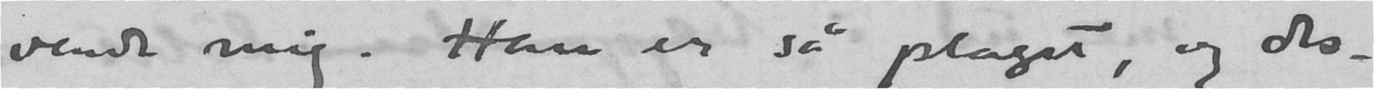vende mig. Han er så plaget, og des-
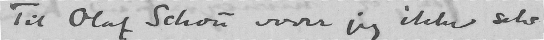Til Olaf Schou vover jeg ikke selv
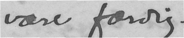være færdig.
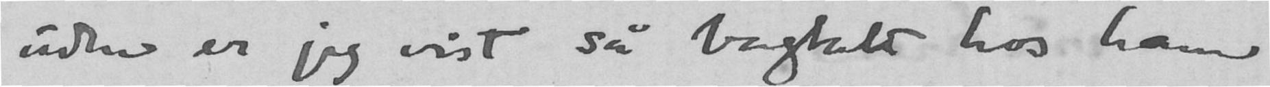uden er jeg vist så bagtalt hos ham
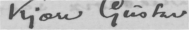Kjære Gustav
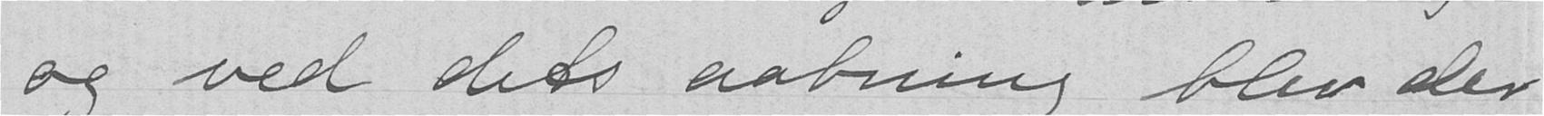og ved dets aabning blev der
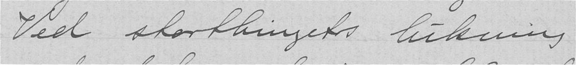Ved storthingets lukning
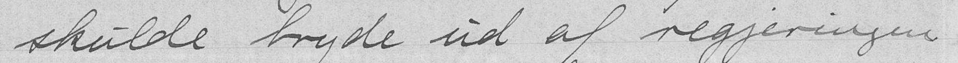skulde bryde ud af regjeringen
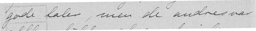gode taler, men de andres var
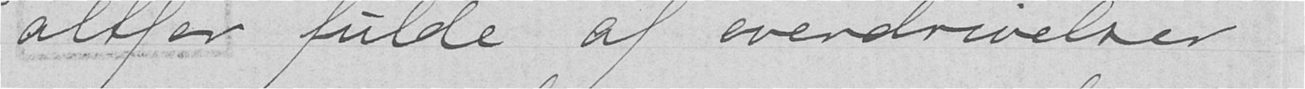altfor fulde af overdrivelser
